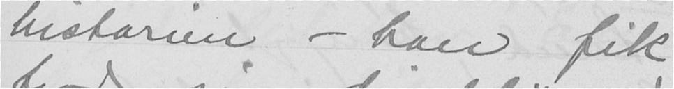historien - han fik
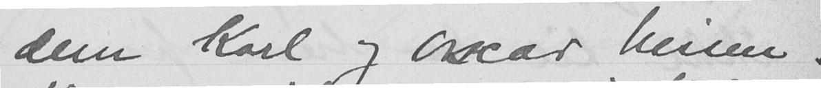dem Karl og Oscar Nissen.
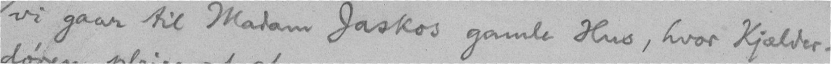vi gaar til Madam Jaskos gamle Hus, hvor Kjælder-
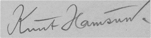Knut Hamsun.
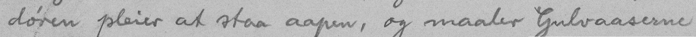døren pleier at staa aapen, og maaler Gulvaaserne
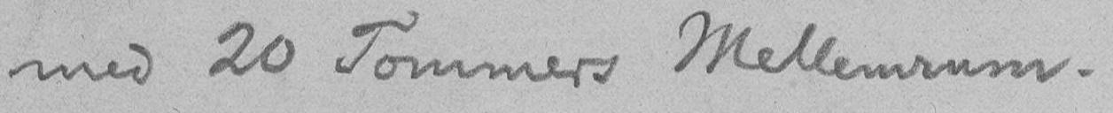med 20 Tommers Mellemrum.
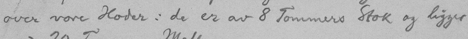over vore Hoder: de er av 8 Tommers Stok og ligger
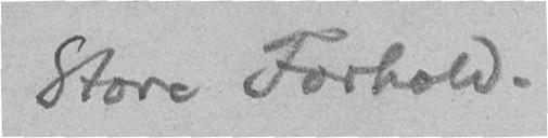Store Forhold.
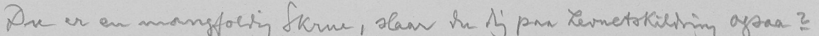Du er en mangfoldig Skrue, slaar du dig paa Levnetskildring ogsaa?
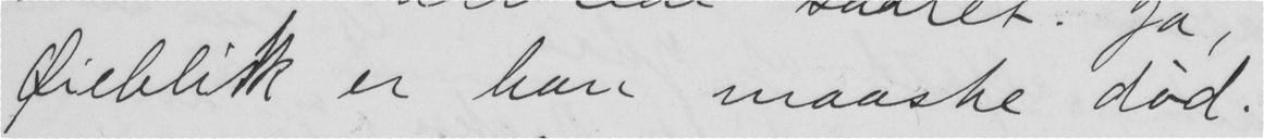Øieblik er han maaske død.
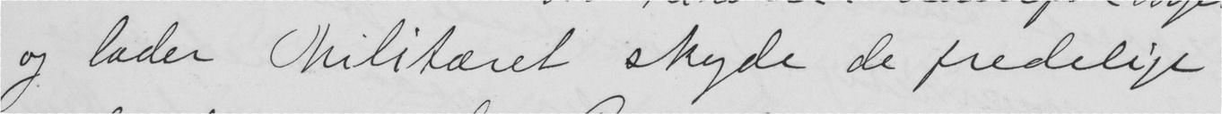og lader Militæret skyde de fredelige
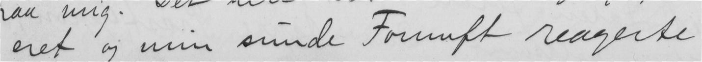eret og min sunde Fornuft reagerte
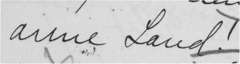arme Land!
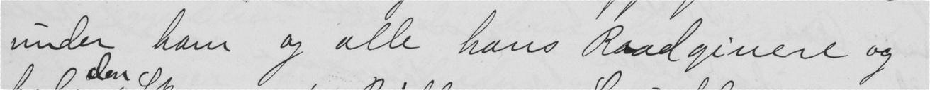under ham og alle hans Raadgivere og
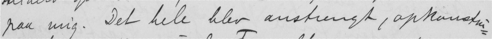paa mig. Det hele blev anstrengt, opkonstru-
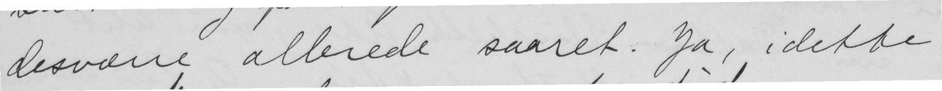desværre allerede saaret. Ja, i dette
